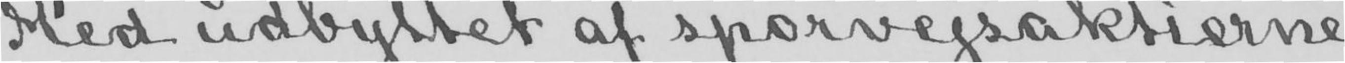Med udbyttet af sporvejsaktierne
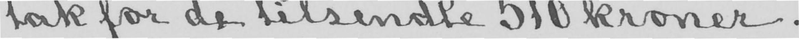tak for de tilsendte 510 kroner.
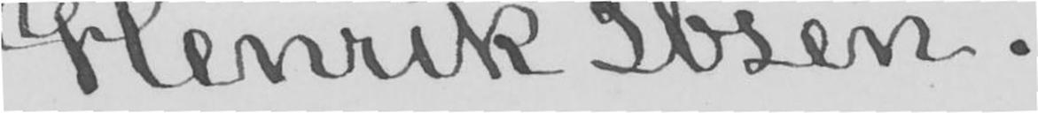Henrik Ibsen.
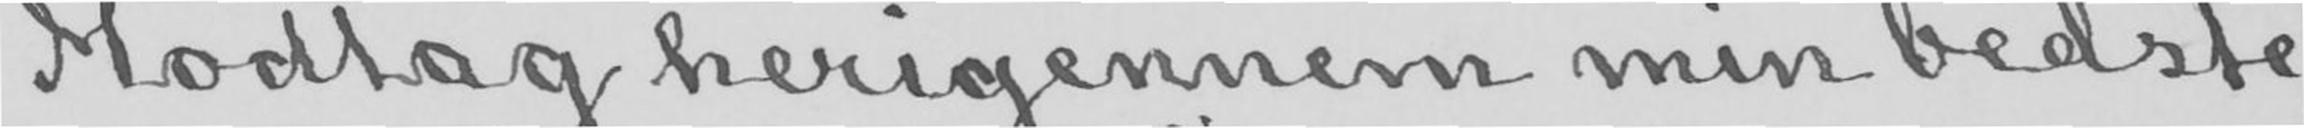Modtag herigennem min bedste
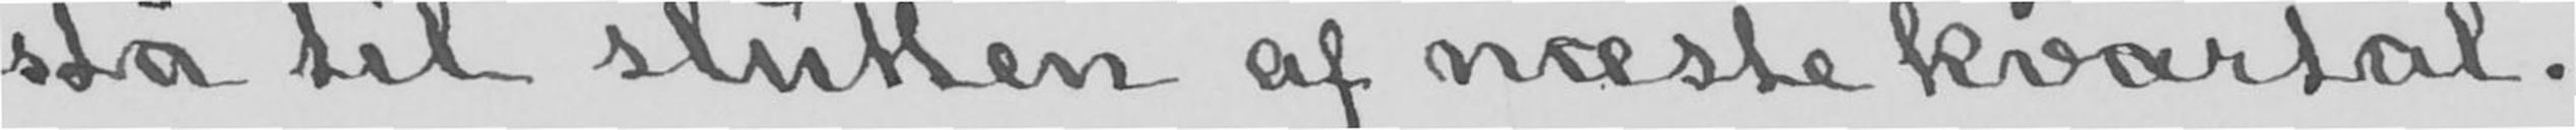stå til slutten af næste kvartal.
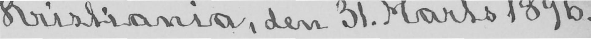Kristiania, den 31. Marts 1896.
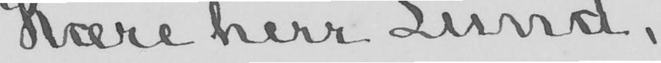Kære herr Lund,
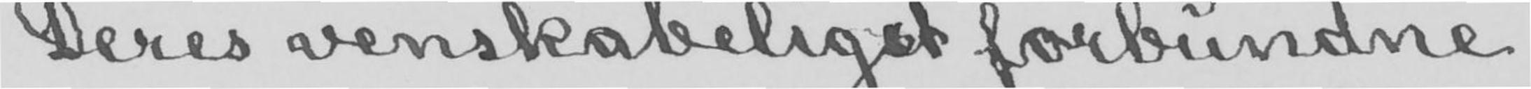Deres venskabeligst forbundne
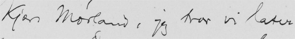Kjære Mørland, jeg tror vi later
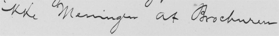ikke Meningen at Brochuren
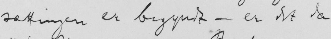sættingen er begyndt - er det da
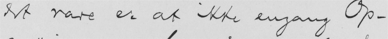det rare er at ikke engang Op-
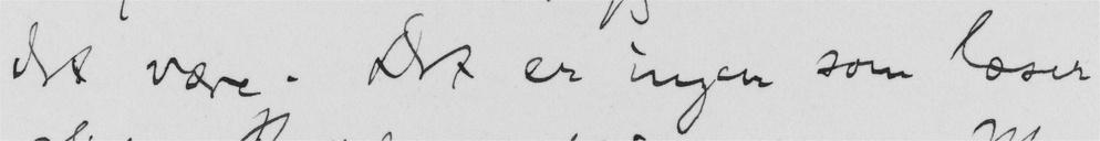det være. Det er ingen som læser
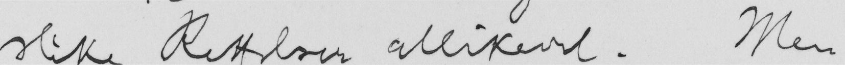slike Rettelser allikevel. Men
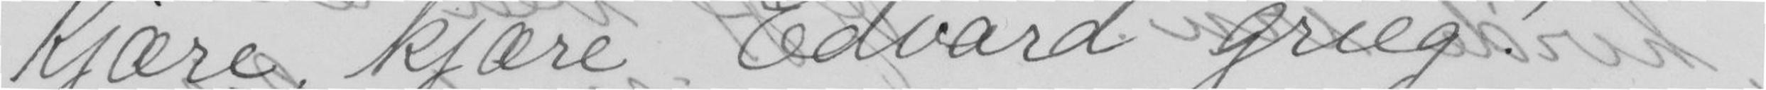Kjære, kjære Edvard Grieg!
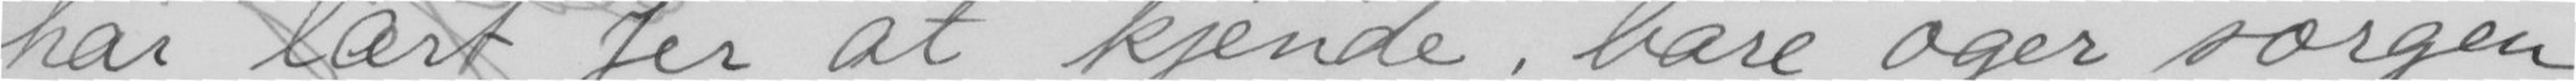har lært Jer at kjende, bare øger sorgen
